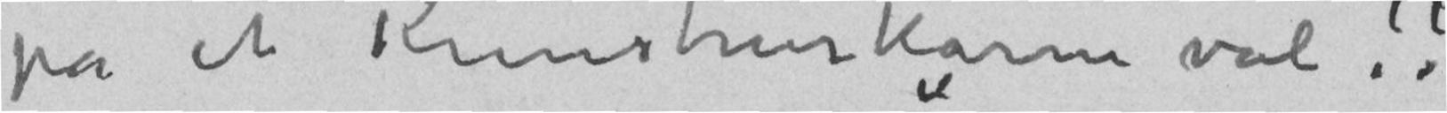på et Kunstnerkarneval!!
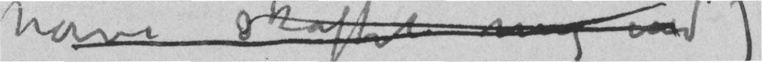have skaffet mig ind)
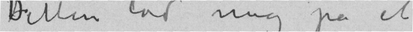Ditten lod mig på et
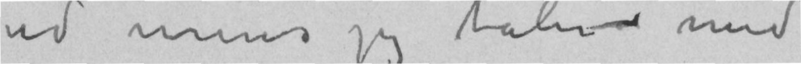ud mens jeg taler med
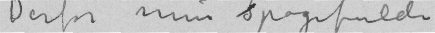Derfor min Sspøgefulde
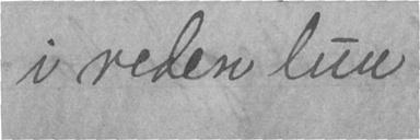i reden lun.
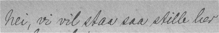Nei, vi vil staa saa stille her
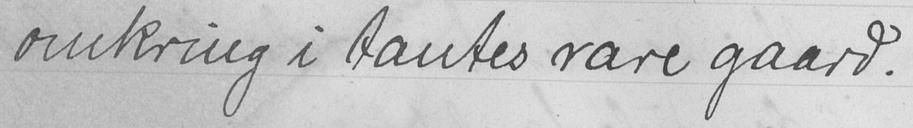omkring i tantes rare gaard.
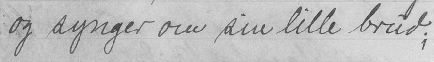og synger om sin lille brud;
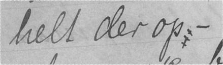helt der op, -
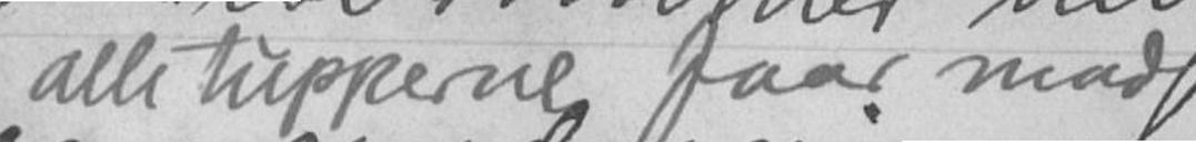alle tupperne faar mad
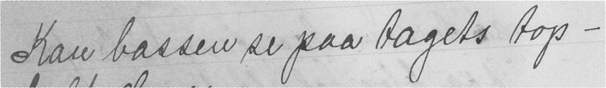Kan bassen se paa tagets top -
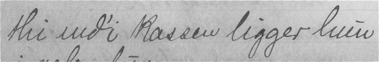thi ind'i kassen ligger hun
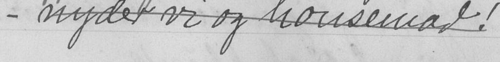nyder vi og hønsemad!
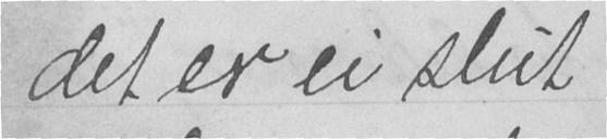det er ei slut
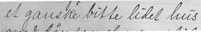et ganske bitte lidet hus
